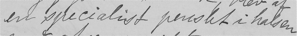en specialist penslet i halsen
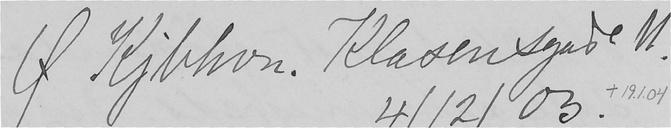Ø Kjbhvn. Klasensgade 11.
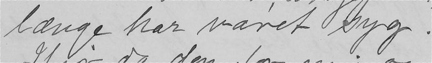længe har været syg.
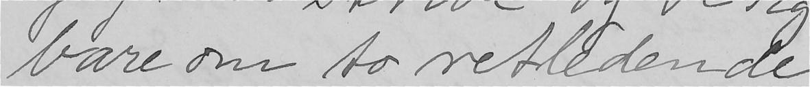bare om to retledende
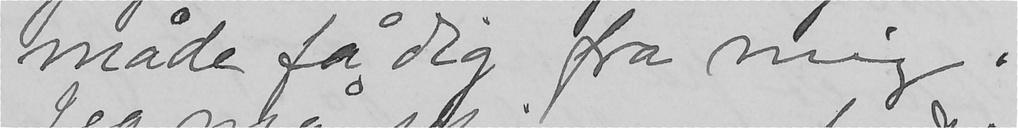måde få dig fra mig.
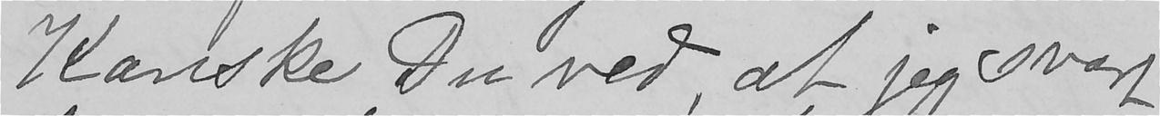Kanske Du ved, at jeg svært
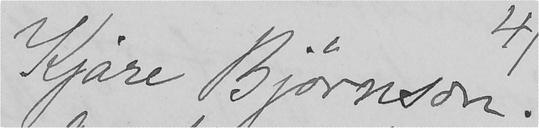Kjære Bjørnson.
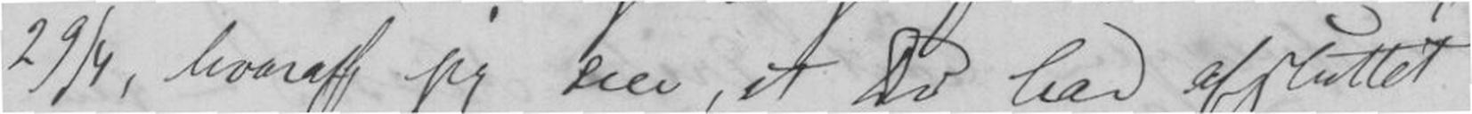29/4, hvoraf jeg seer, at Du har afsluttet
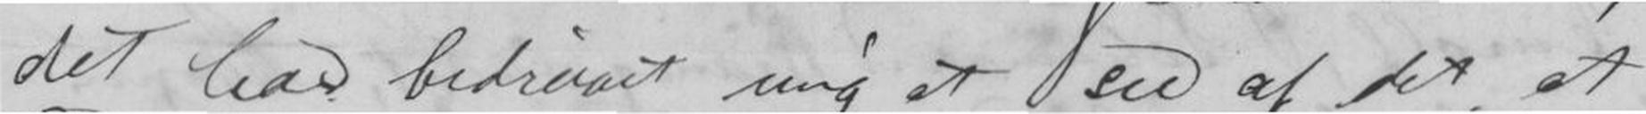det har bedrøvet mig at see af det, at
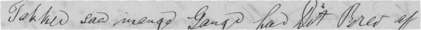Takker saa mange Gange for Dit Brev af
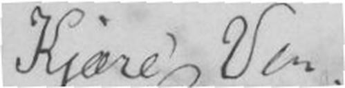Kjære Ven!
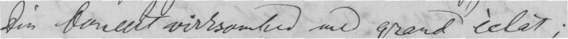Din Concert virksomhed med grand cèlat;
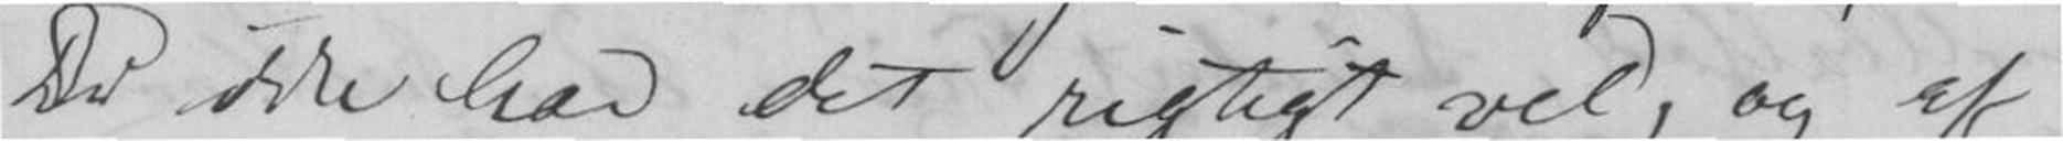Du ikke har det rigtigt vel, og af
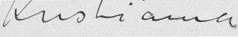Kristiania
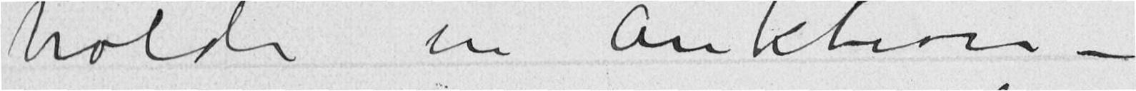holde en Auktion –
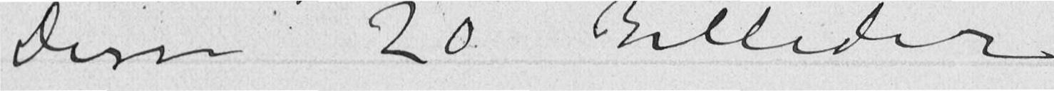Disse 20 Billeder
